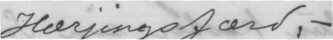Hærjingsfærd, -
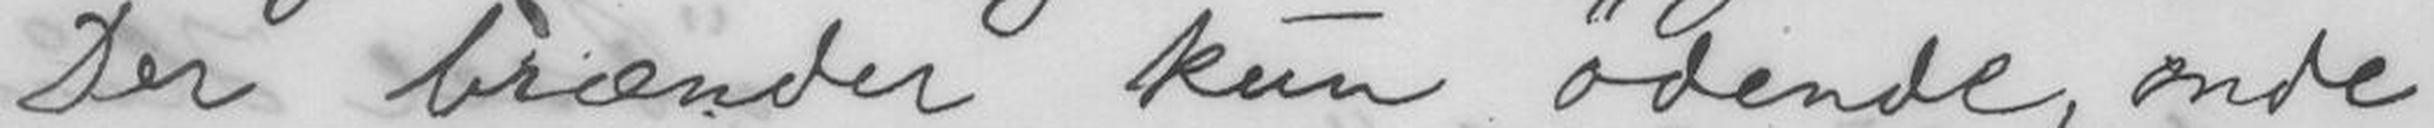Der brænder kun ødende, onde
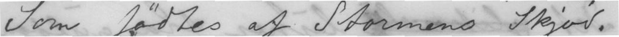Som fødtes af Stormens Skjød.
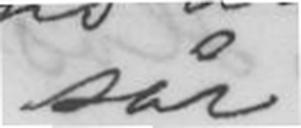sår
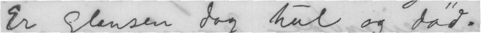Er glansen dog hul og død!
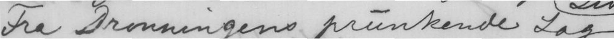Fra Dronningens Prunkende Lag,
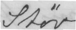Støv.
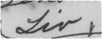Liv,
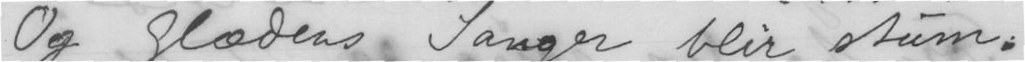Og Glædens Sanger blir stum.
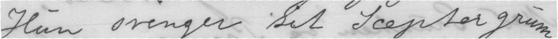Hun svinger sit Sæpter grunn,
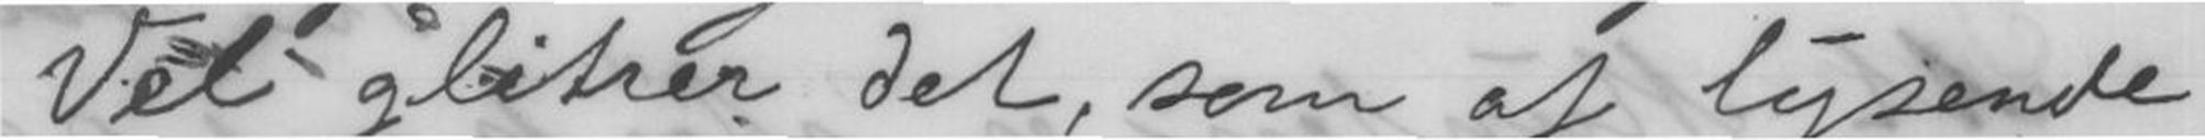Vel glitrer det, som af lysende
